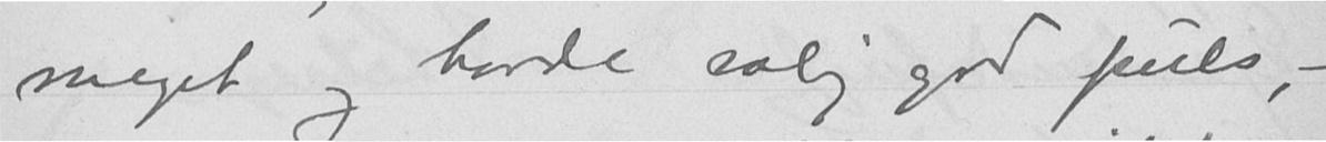meget og havde salig god puls, -
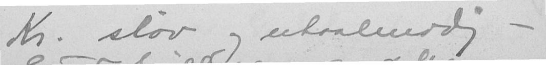Kr. sløv og utaalmodig -
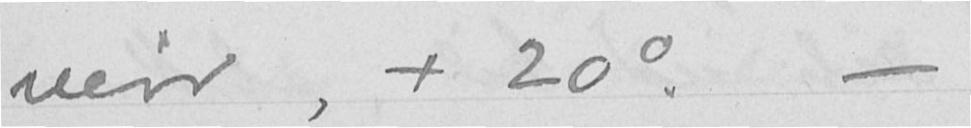veir, + 20°. -
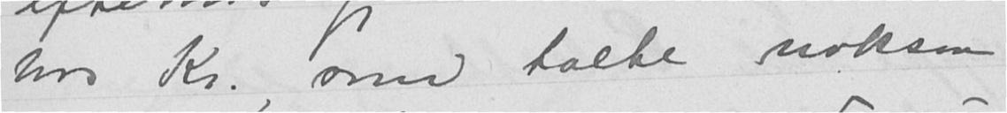hos Kr., som talte noksaa
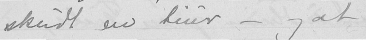skudt en tiur - og at
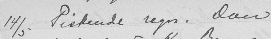14/5 Piskende regn. Don
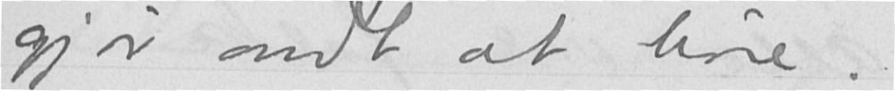gjør ondt at høre.
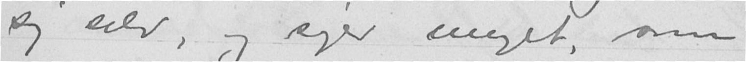sig selv, og siger meget, som
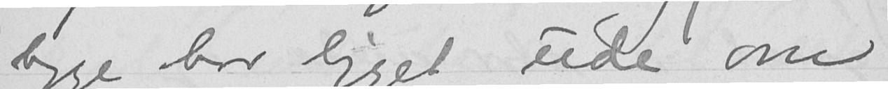begge har ligget ude om
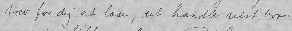brev for dig at læse; det handler vist bare
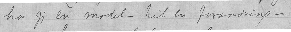har jeg en model — til en forandring —
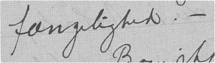fængelighed. —
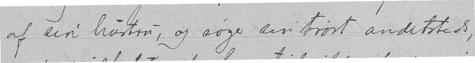af sin hustru —, og søger sin trøst andetsteds,
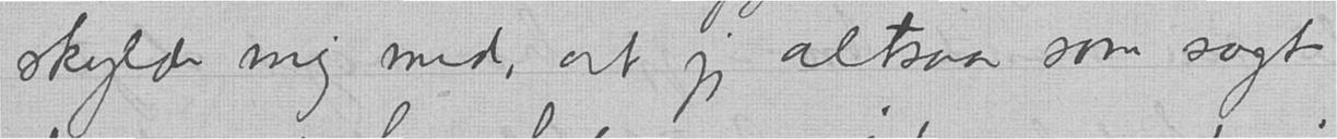skylde mig med, at jeg altsaa som sagt
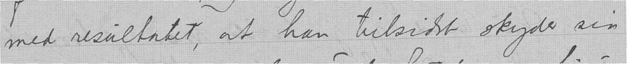med resultatet, at han tilsidst skyder sin
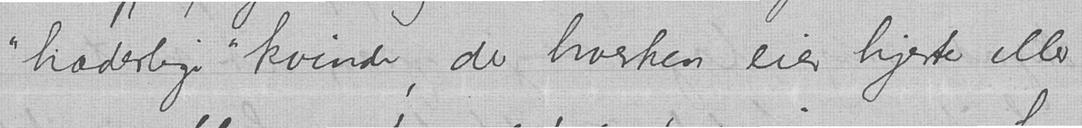"hæderlige" kvinde, der hverken eier hjerte eller
